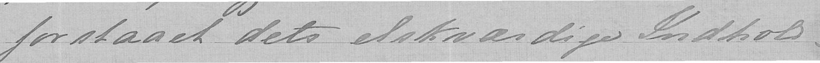forstaaet dets elskværdige Indhold.
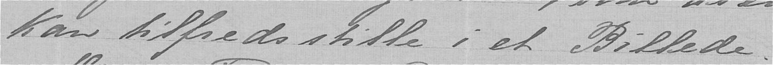kan tilfredsstille i et Billede.
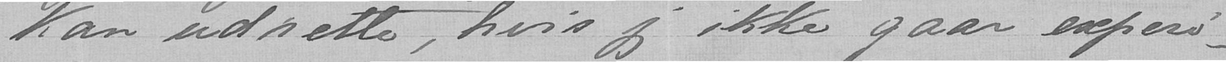kan udrette, hvis jeg ikke gaar experi-
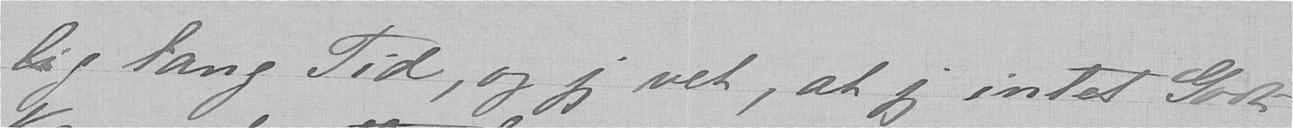lig lang Tid, og jeg vet, at jeg intet Godt
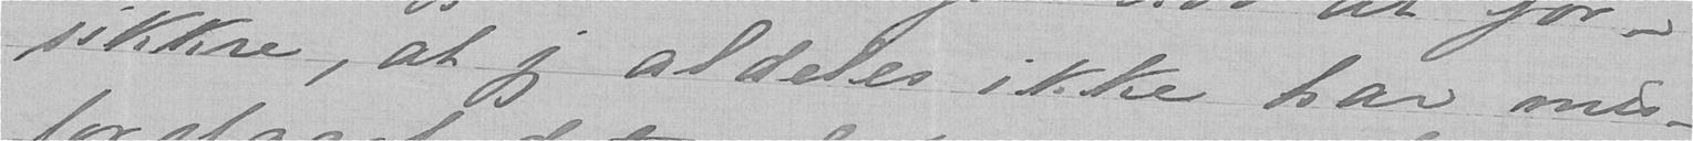sikkre, at jeg aldeles ikke har mis-
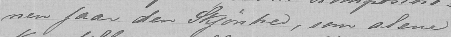nen faar den Skjønhed, som alene
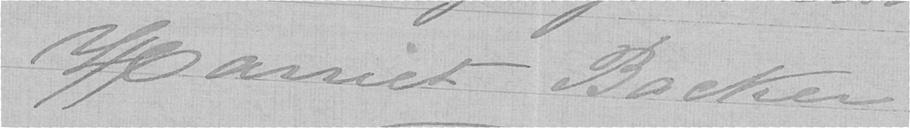Harriet Backer
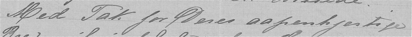Med Tak for Deres aapenhjertige
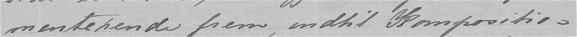menterende frem indtil Kompositio-
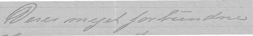Deres meget forbundne
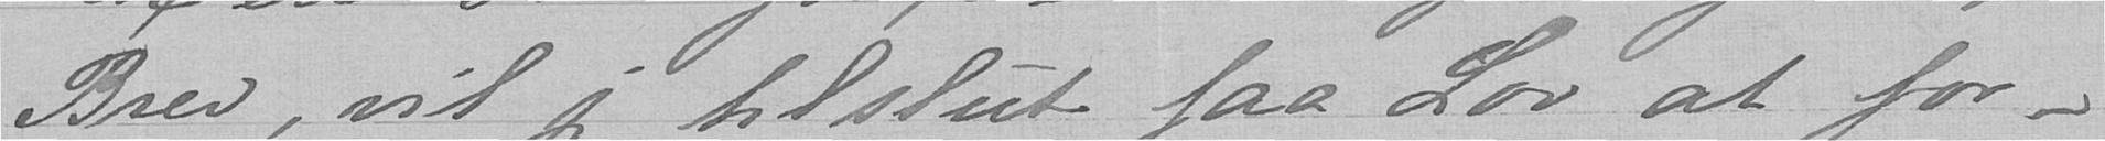Brev, vil jeg tilslut faa Lov at for-
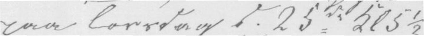paa Torsdag d. 25de kl. 5 1/2.
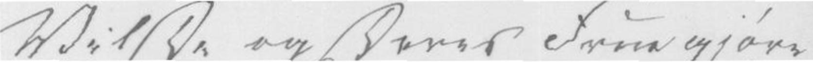Vil De og Deres Frue gjøre
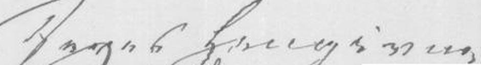Deres hengivne
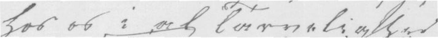hos os i al Tarvelighed
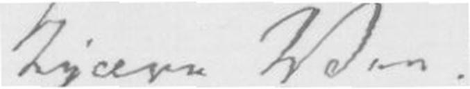Kjære Ven!
